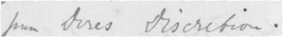paa Deres Discretion.
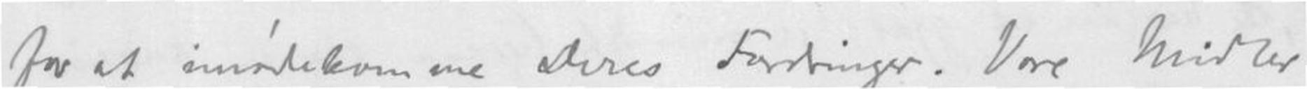for at imødekomme Deres Fordringer. Vore Midler
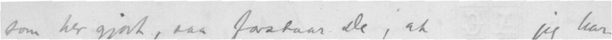som her gjort, saa forstaar De, at jeg har
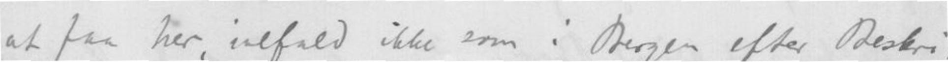at faa her, ialfald ikke som: Bergen efter Beskri
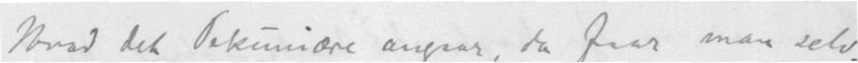Hvad det pekuniære angaar, da faar man selv
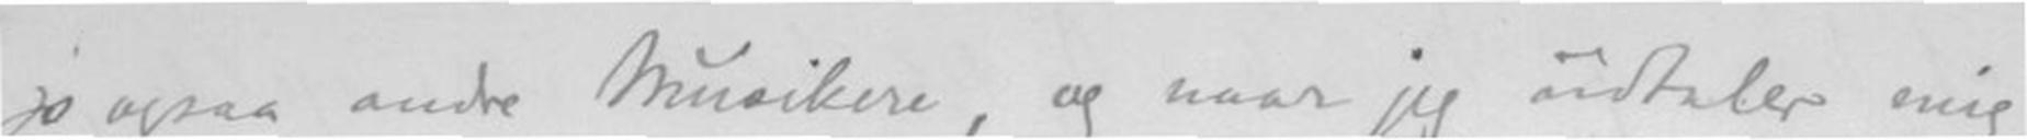jo ogsaa andre Musikere, og naar jeg udtaler mig
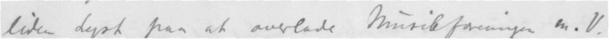liden lyst paa at overlade Musikforeningen m.V.
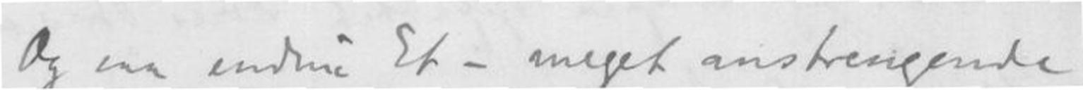Og saa endnu Et - meget anstrengende
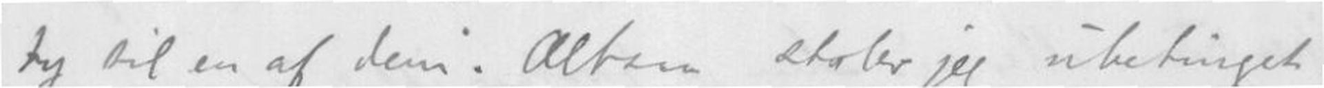ty til en af dem. Altsaa stoler jeg ubetinget
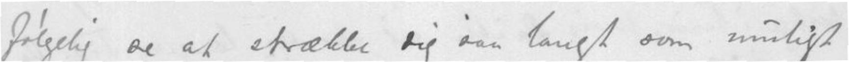følgelig se at strække sig saa langt som muligt
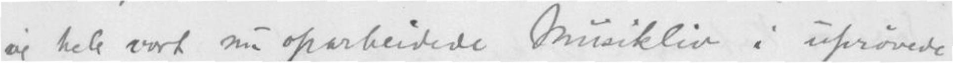j. hele vort nu oparbeidede Musikliv i uprøvede
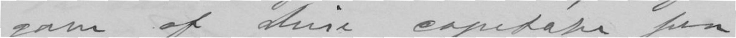gave af disse copetape som
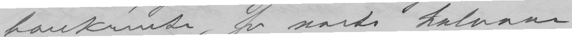bankrente, for næste halvaar
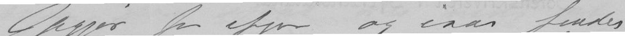Opgjør for ifjor og iaar sendes
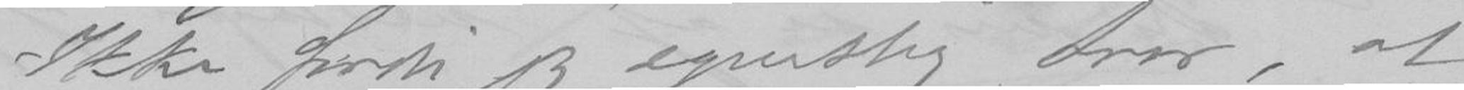Ikke fordi jeg egentlig tror, at
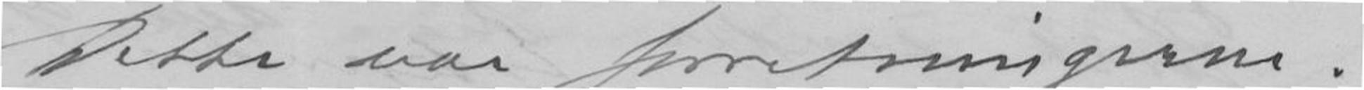Dette var forretningerne.
